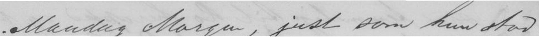Mandag Morgen, just som hun stod
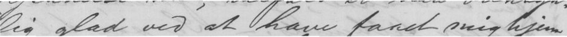lig glad ved at have faaet mig hjem,
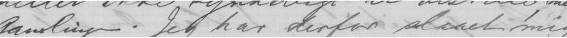Gamlingen. Jeg har derfor slaaet mig
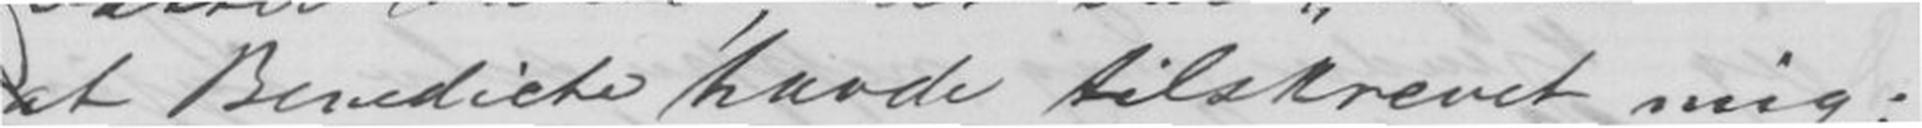at Benedicte havde tilskrevet mig;
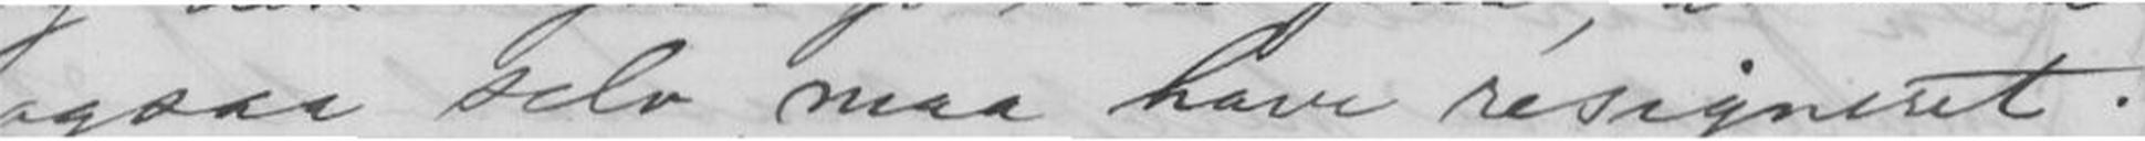ogsaa selv maa have resigneret.
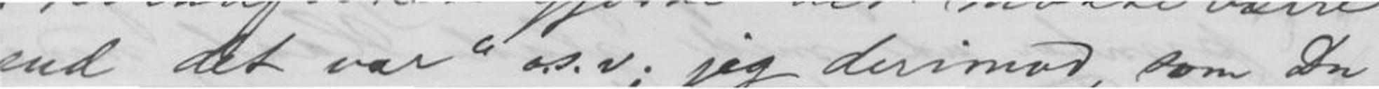end det var o.s.v; jeg derimod, som Du
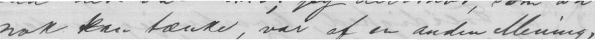nok kan tænke, var af en anden Mening,
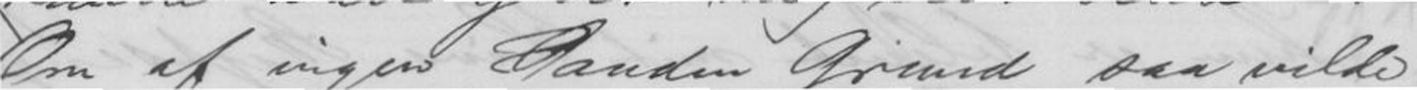Om af ingen anden Grund, saa vilde
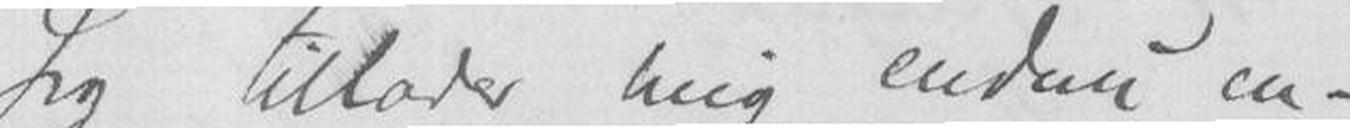Jeg tilader mig endnu en-
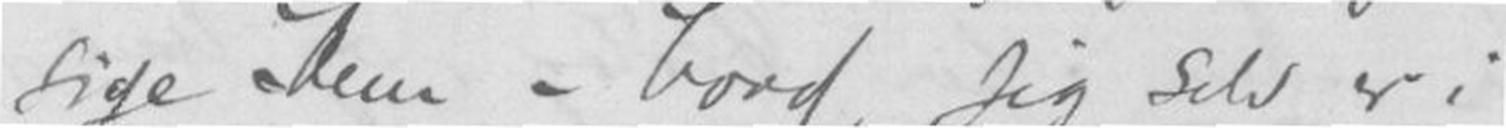Dem - bord jeg selv er i
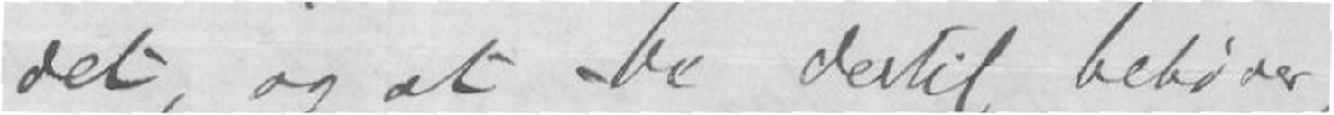det, og at det dertil behøver
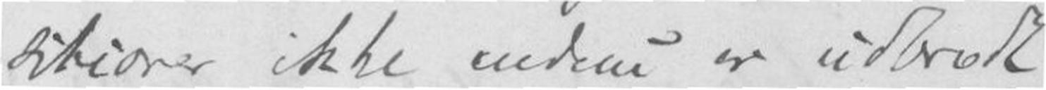sitioner ikke endnu er udbrædt
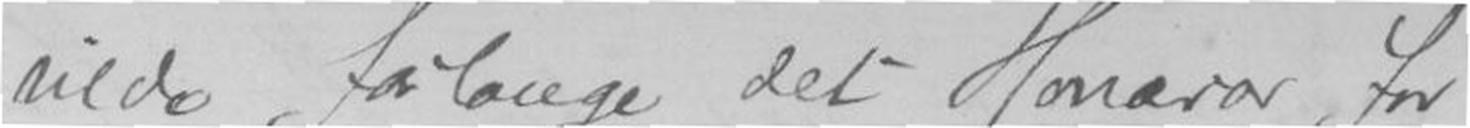vilde forlange det Honorar for
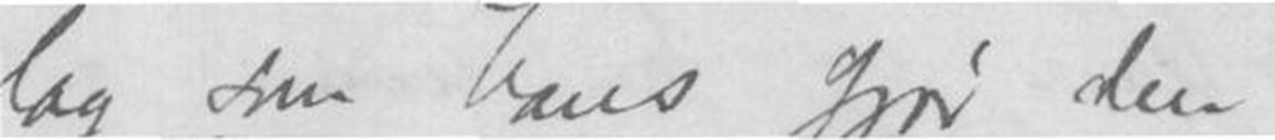lag som hans gjør den
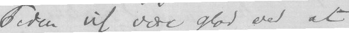Tiden vil være glad over at
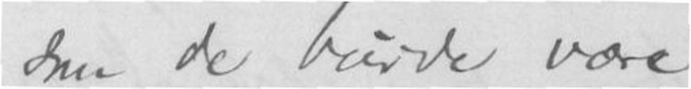men de burde være
